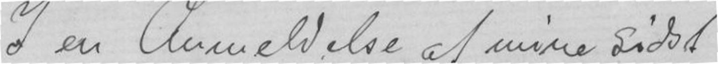I en Anmeldelse af mine sidst
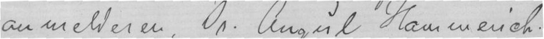anmelderen, Dr. Angul Hammerich.
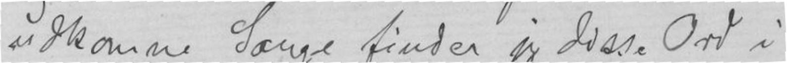udkomne Sange finder jeg disse Ord i
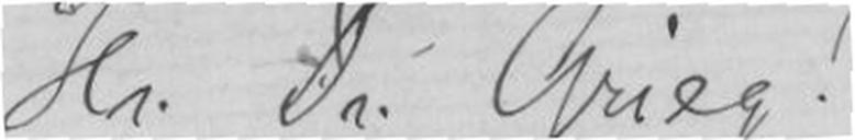Hr Dr. Grieg!
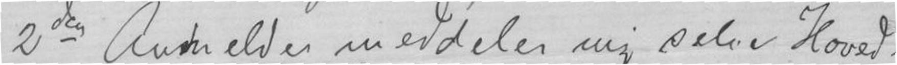2den Anmelder meddeler mig selve Hoved-
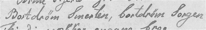Bortdrøm Smerten, bortdrøm Sorgen
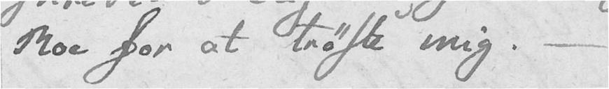Roe for at trøste mig. –
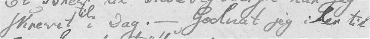skrevet i Dag. – Godnat jeg iler til
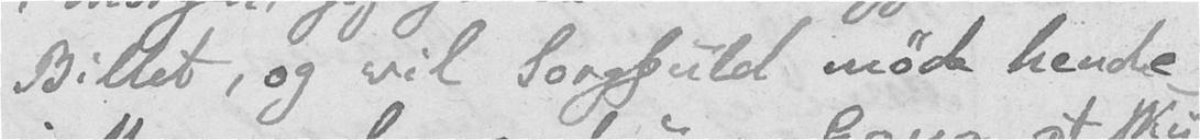Billet, og vil Sorgfuld møde hende
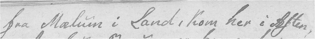fra Mælum i Land, kom her i Aften,
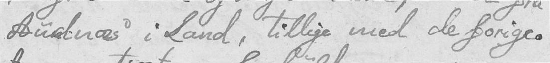Audnæs i Land, tillige med de forige.
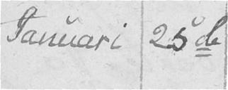Januari 25de
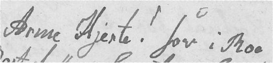Arme Hjerte! sov i Roe
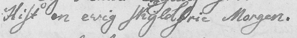Hist en evig skyldfrie Morgen!
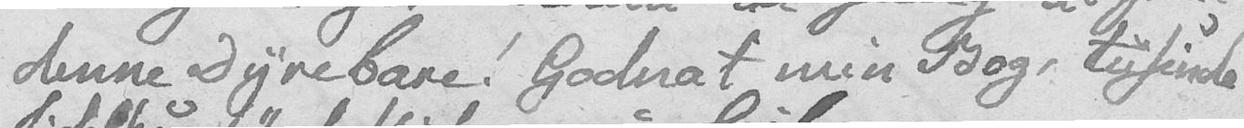denne Dyrebare! Godnat min Bog, tusinde
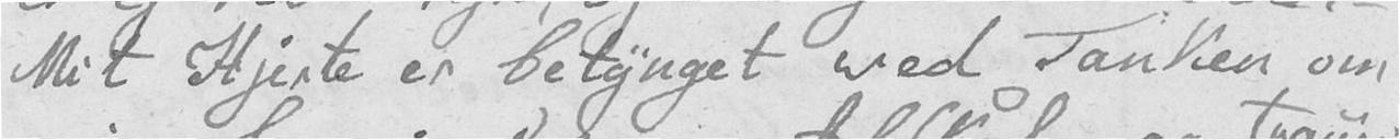Mit Hjerte er betynget ved Tanken om
